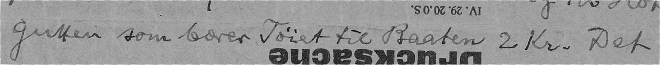gutten som bærer Tøiet til Baaten 2 Kr. Det
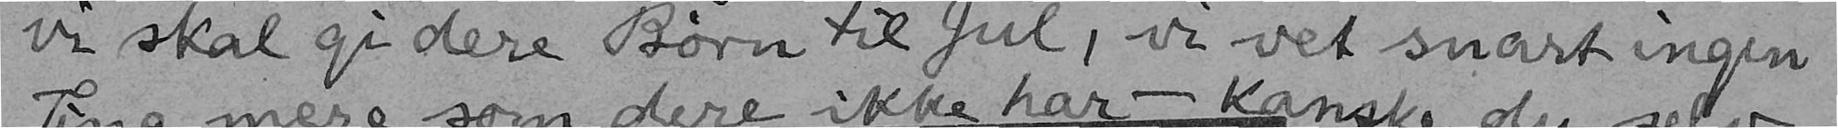vi skal gi dere Børn til Jul, vi vet snart ingen
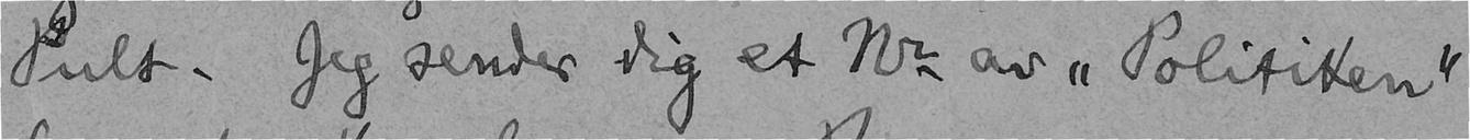Pult. Jeg sender dig et Nr av "Politiken"
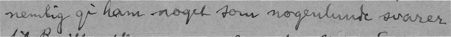nemlig gi ham noget som nogenlunde svarer
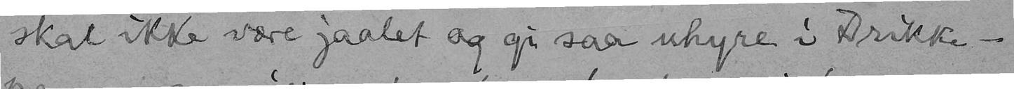skal ikke være jaalet og gi saa uhyre i Drikke-
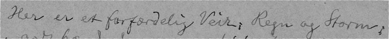Her er et forfærdelig Veir; Regn og Storm;
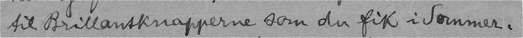til Brillantknapperne som du fik i Sommer.
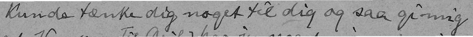kunde tænke dig noget til dig og saa gi mig
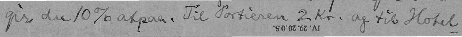gir du 10% atpaa. Til Portieren 2 Kr. og til Hotel-
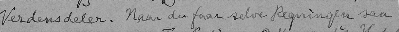Verdensdeler. Naar du faar selve Regningen saa
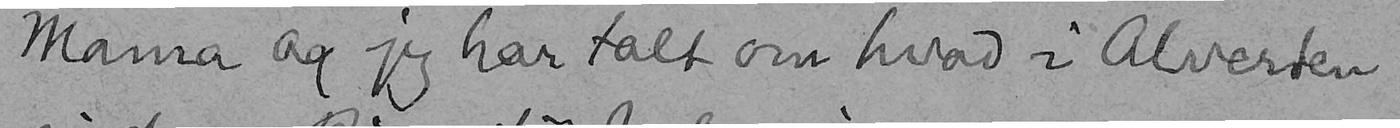Mama og jeg har talt om hvad i Alverden
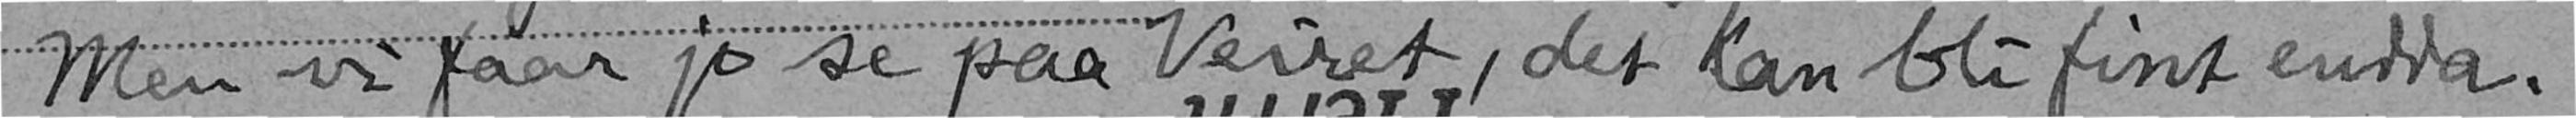Men vi faar jo se paa Veiret, det kan bli fint endda.
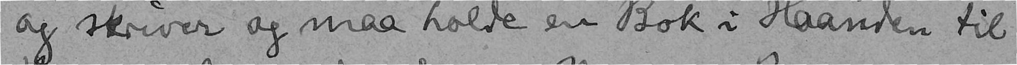og skriver og maa holde en Bok i Haanden til
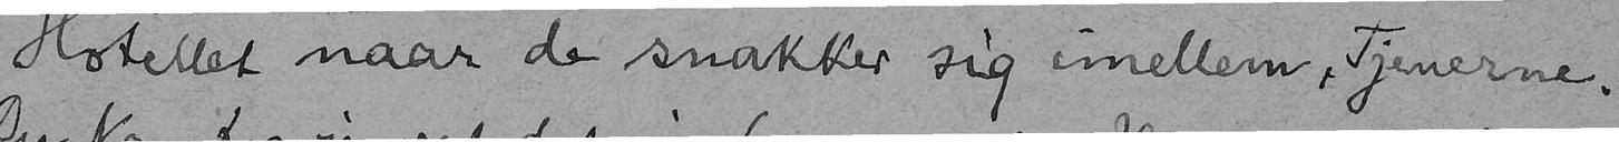Hotellet naar de snakker sig imellem, Tjenerne.
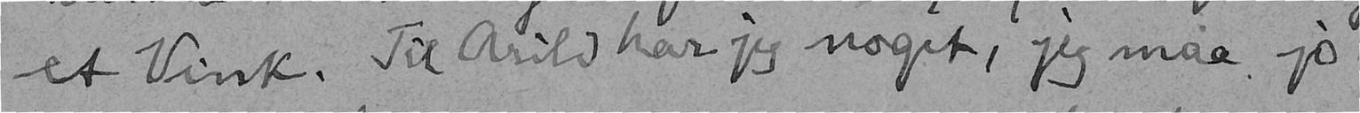et Vink. Til Arild har jeg noget, jeg maa jo
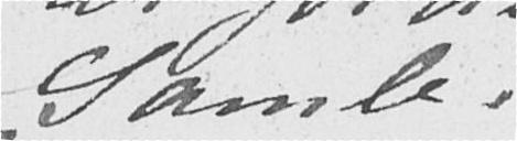Gamle.
